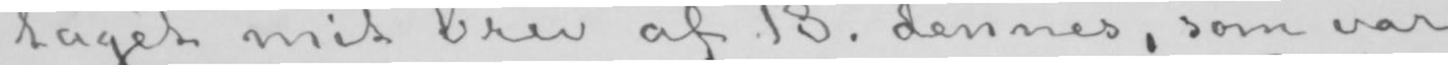taget mit brev af 18. dennes, som var
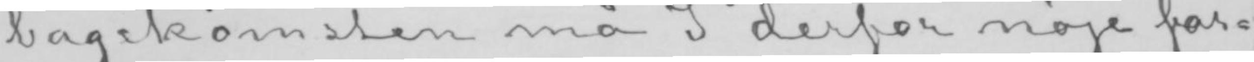bagekomsten må I derfor nøje for-
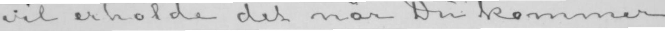vil erholde det når Du kommer
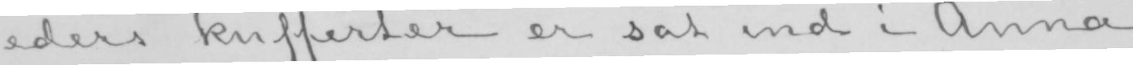eders kufferter er sat ind i Anna
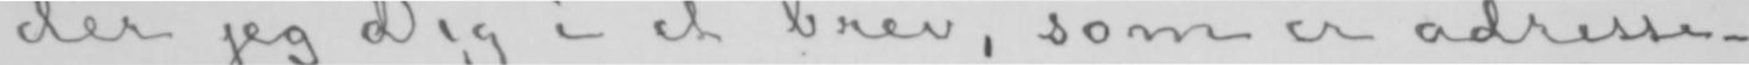der jeg Dig i et brev, som er adresse-
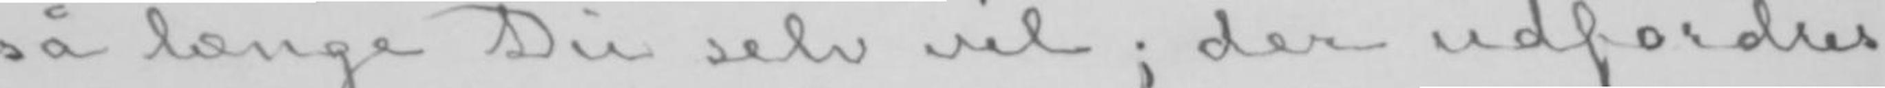så længe Du selv vil; der udfordres
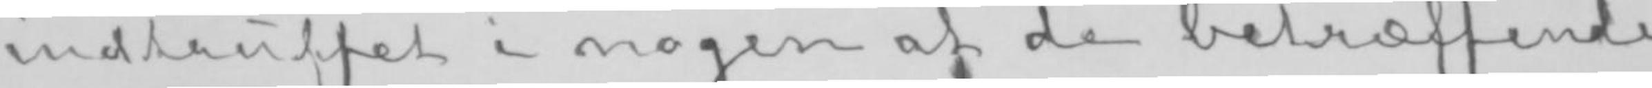indtruffet i nogen af de betræffende
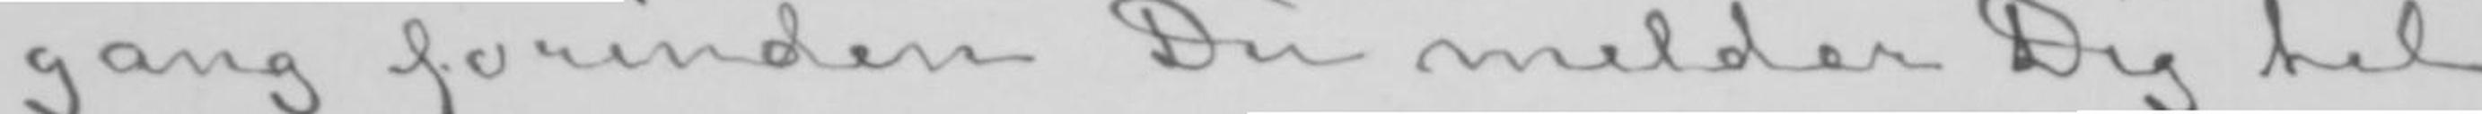gang forinden Du melder Dig til
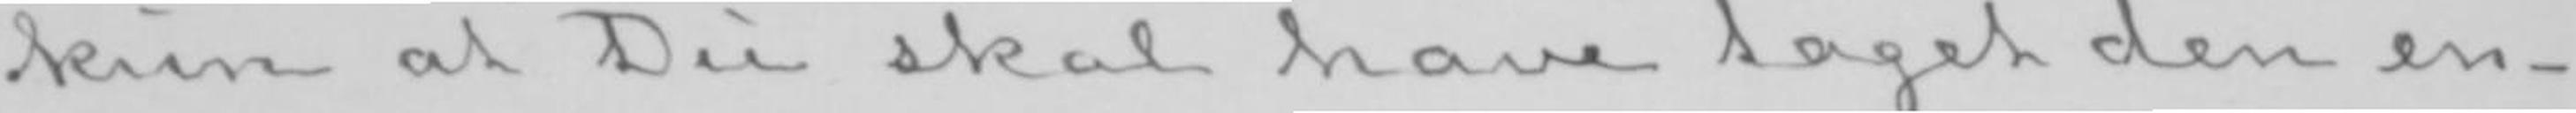kun at Du skal have taget den en-
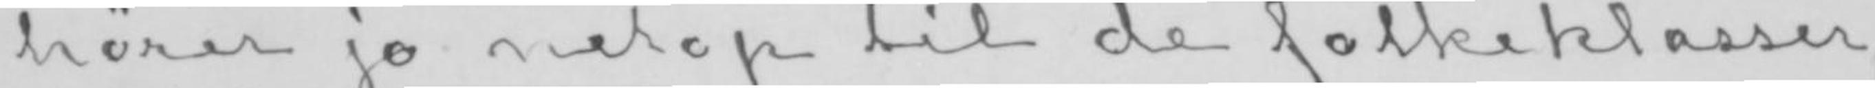hører jo netop til de folkeklasser,
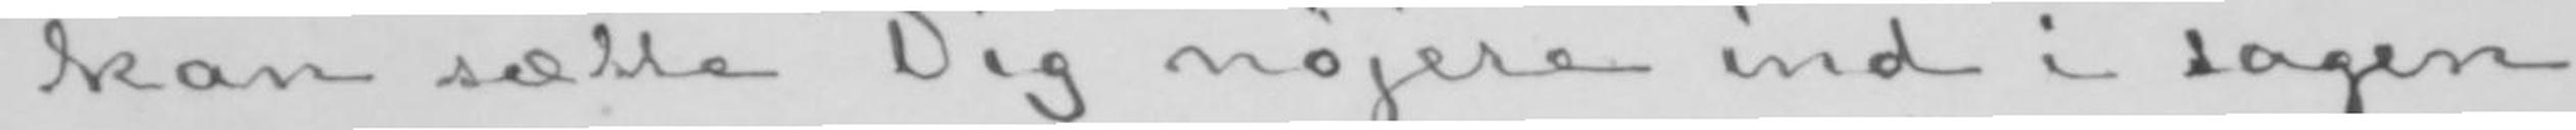kan sætte Dig nøjere ind i sagen
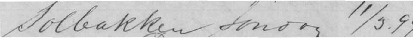Solbakken Søndag 11/3. 94.
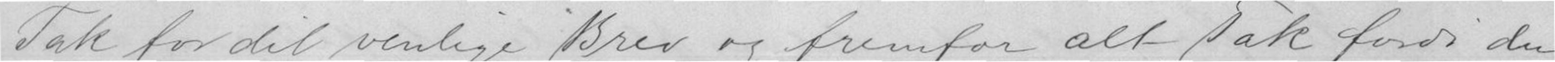Tak for dit venlige Brev og fremfor alt, Tak fordi du
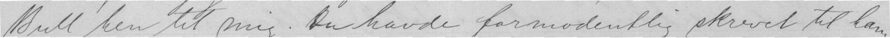Bull hen til mig. Du havde formodentlig skrevet til ham
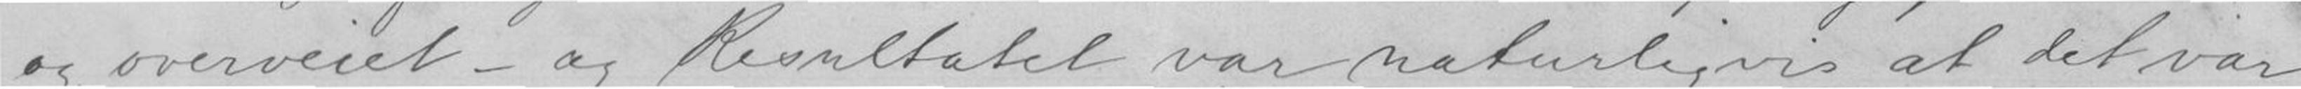og overveiet - og Resultatet var naturligvis at det var
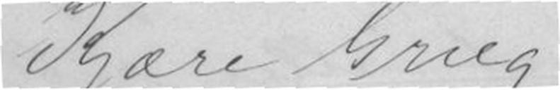Kjære Grieg
